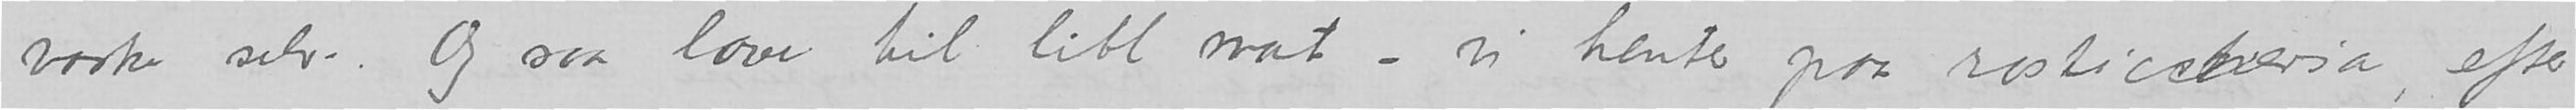vasker selv. Og saa laver til litt mat – vi henter paa rosticceria, efter
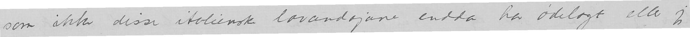som ikke disse italienske lavandajane endda har ødelagt eller jeg
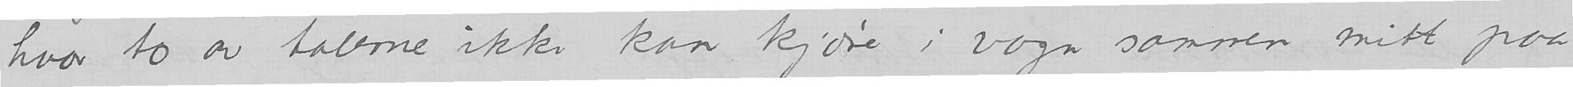hvor to av talerne ikke kan kjøre i vogn sammen midt paa
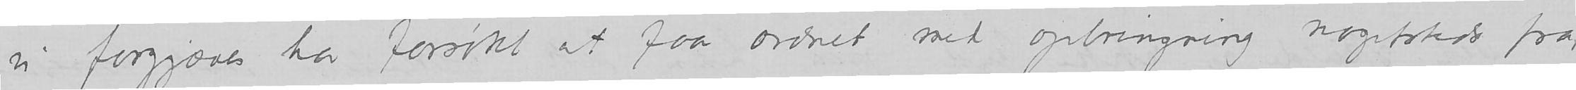vi forgjæves har forsøkt at faa ordnet med opbringning nogetsteds fra,
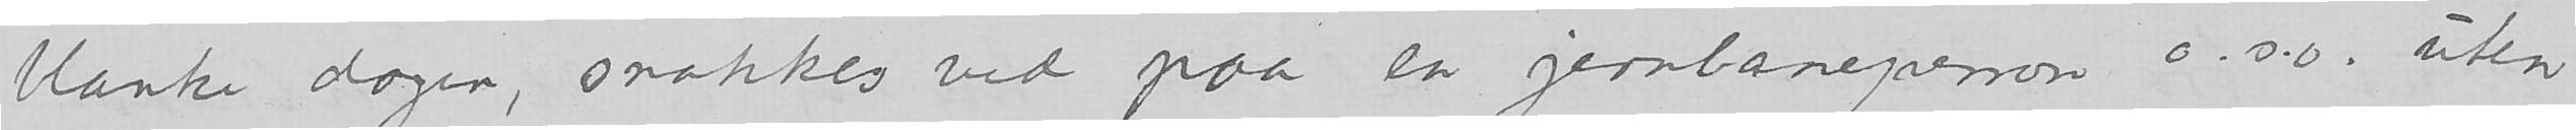blanke dagen, snakkes ved paa en jernbaneperron o.s.v. uten
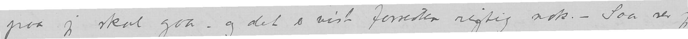paa jeg skal gaa – og det er vist forresten rigtig nok. – Saa ser jeg
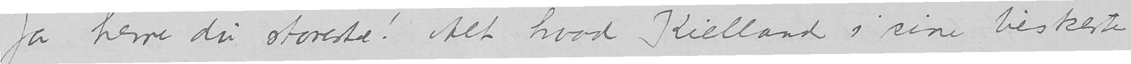Ja herre du storeste! Alt hvad Kielland i sine biskeste
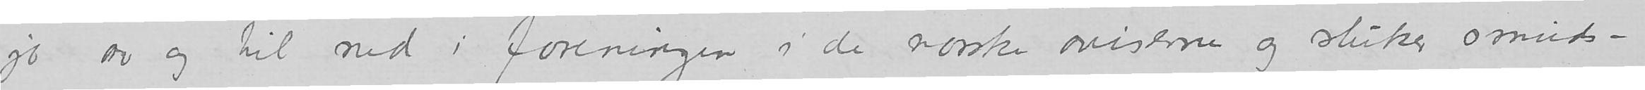jo av og til ned i foreningen i de norske aviserne og sluker smuds –.
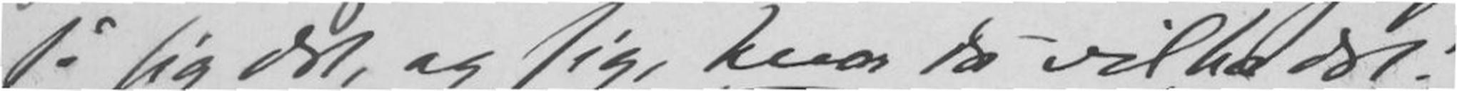så sig det, og sig, hvor du vil ha det!
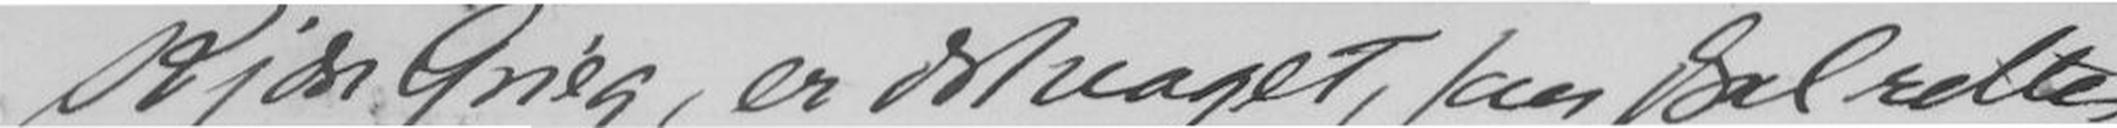Kjære Grieg, er det noget, som skal rettes,
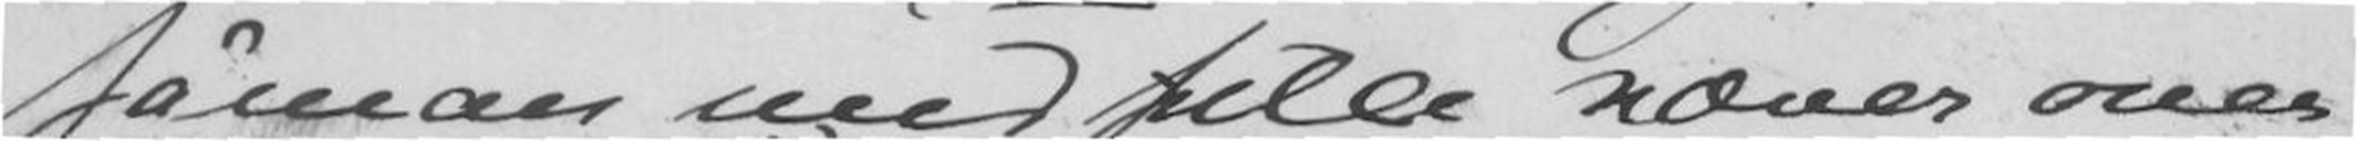såman med fulle næver over
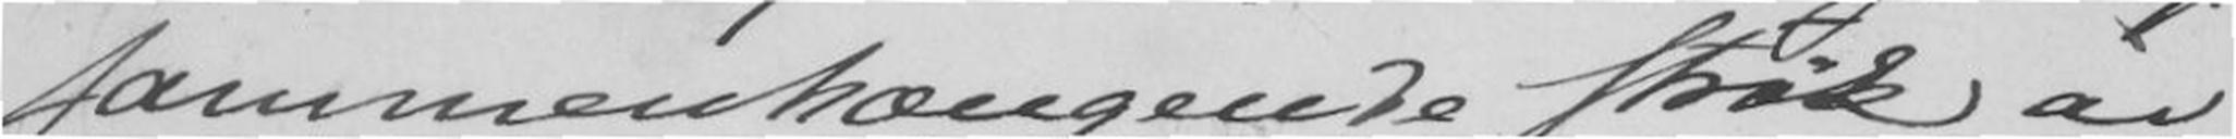sammenhængende strøk av
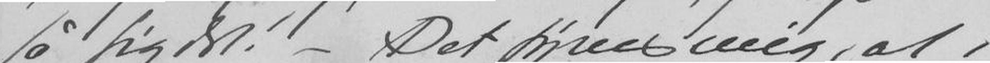så sig det! - Det synes mig, at i
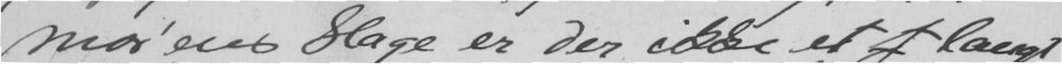mor'ens klage er der ikke et s langt
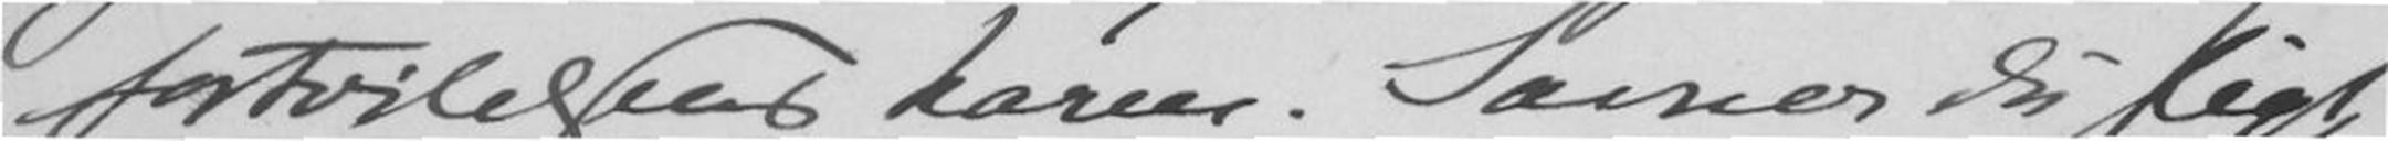fortvilelsens harm. Savner du sligt
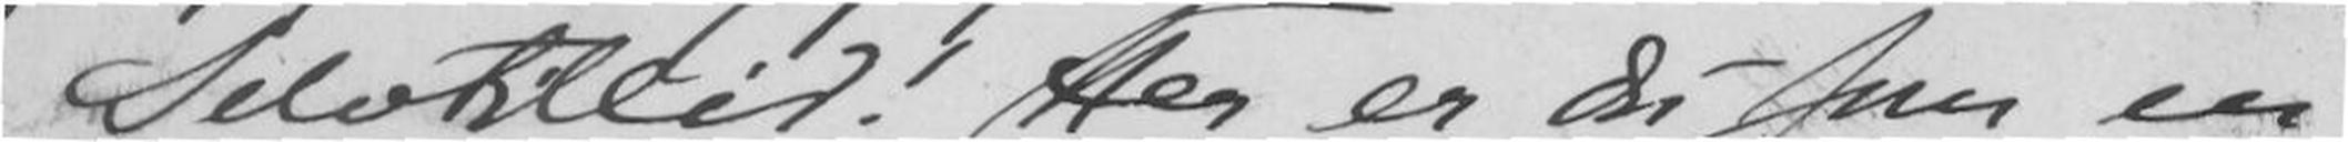Selvtillid! Her er du som en
